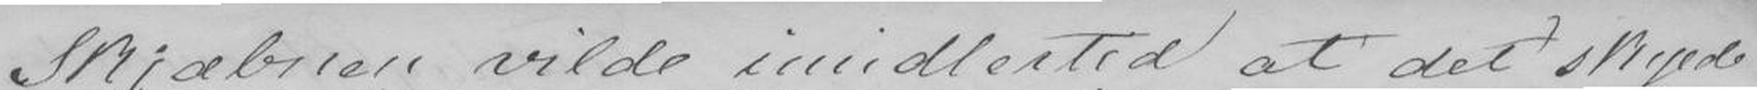Skjæbnen vilde imidlertid at det skyede
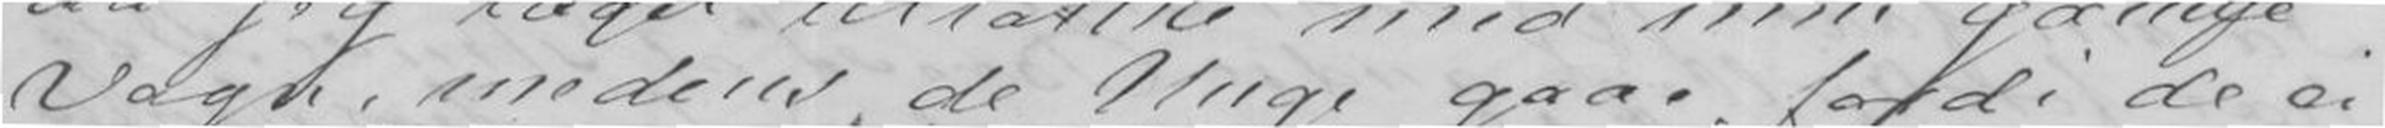Vogn, medens de Unge gaaer fordi de ei
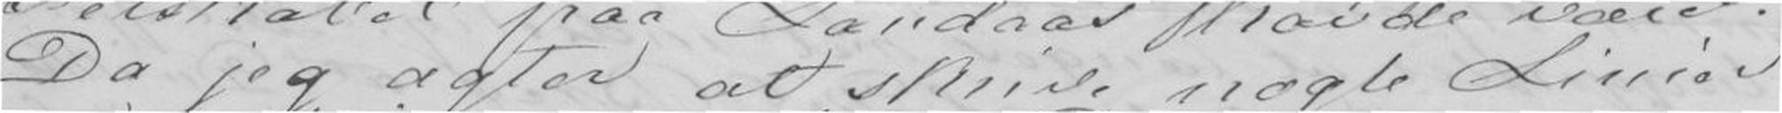Da jeg agter at skrive nogle Linier
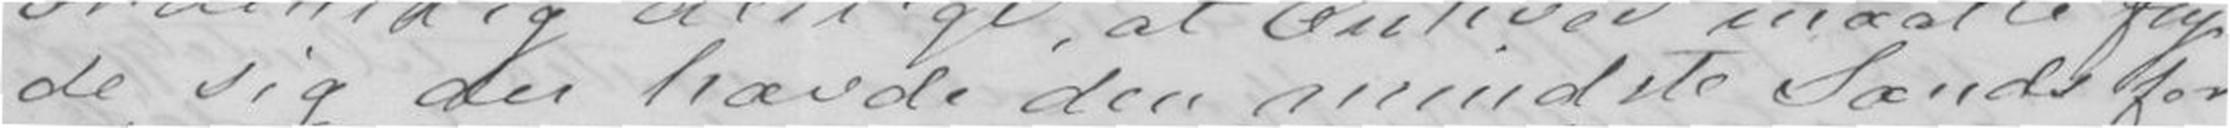de sig der havde den mindste Sands for
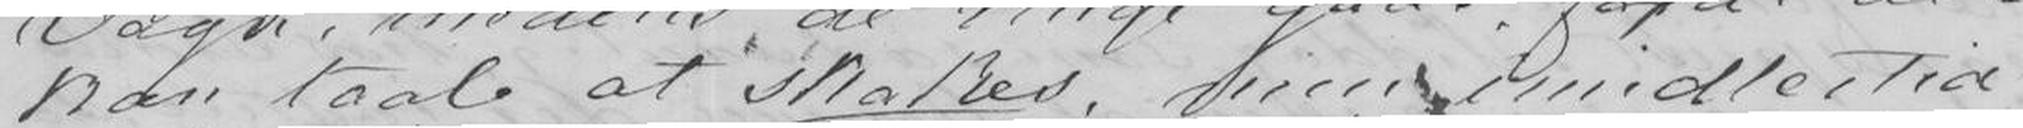kan taales at skakes, men imidlertid
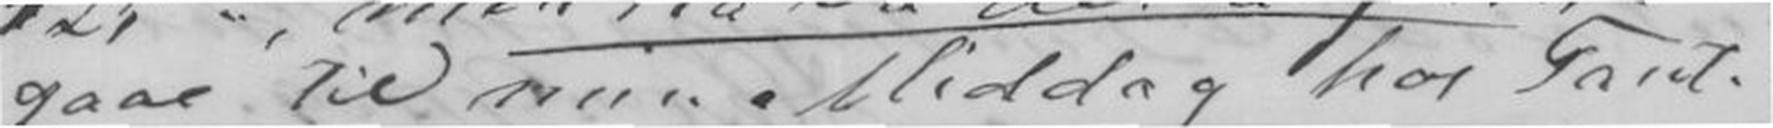gaae til min Middag hos Tante
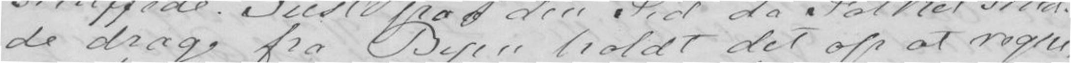de drage fra Byen holdt det op at regne,
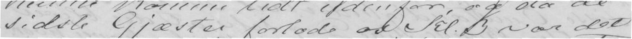sidste Gjæster forlade os Kl.3 var det
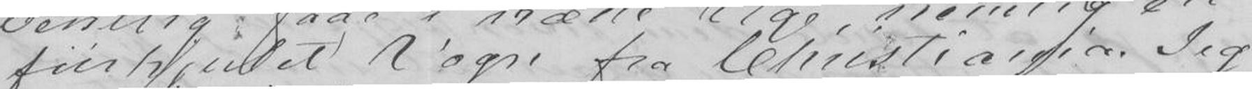fiirhjulet Vogn fra Christiania. Jeg
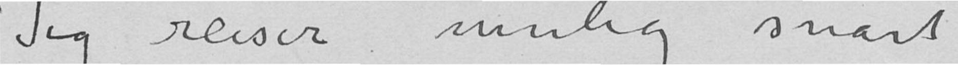Jeg reiser nemlig snart
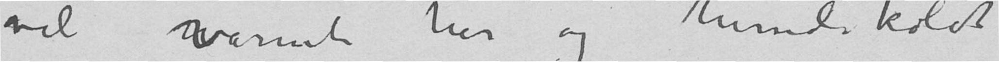vel varmt her og hundekoldt
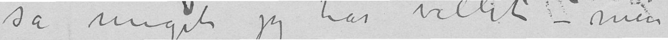så meget jeg har villet – men
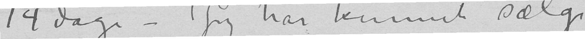14 dage – Jeg har kunnet sælge
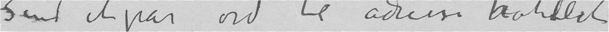Send etpar ord til adresse hotellet
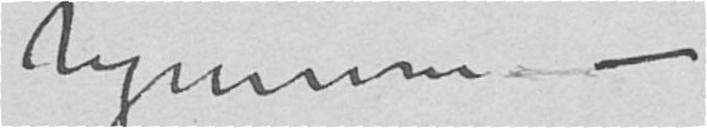hjemme –
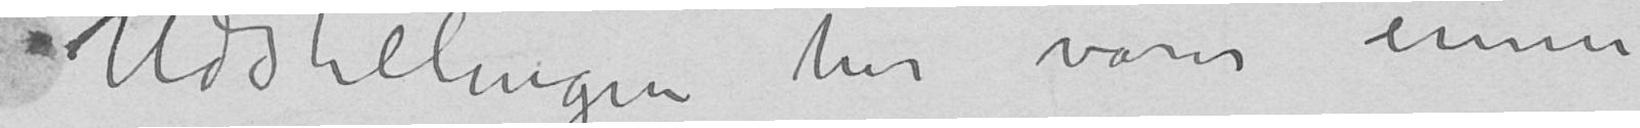Udstillingen her varer ennu
